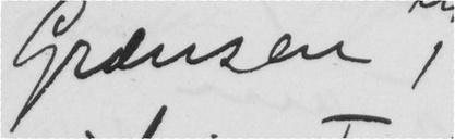Grænsen,
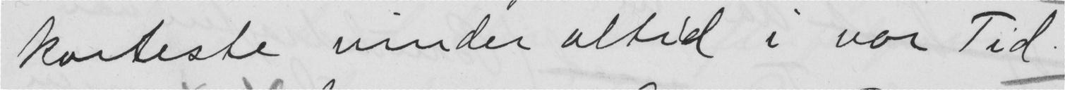korteste vinder altid i vor Tid.
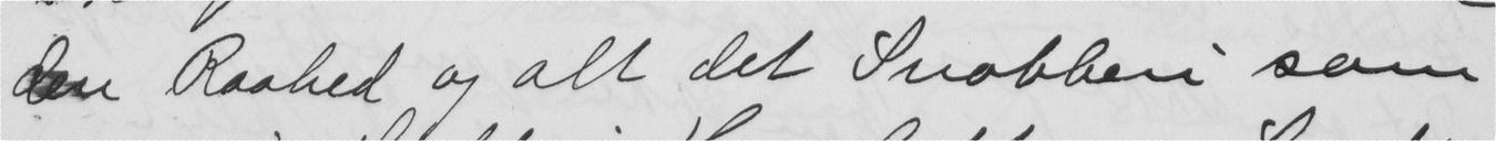den Raahed og alt det Snobberi som
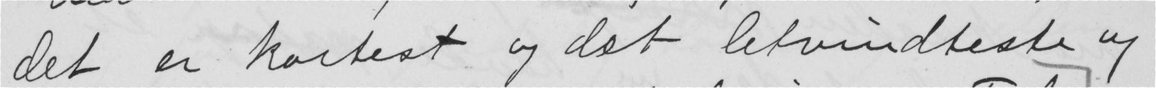det er kortest og det letvindteste og
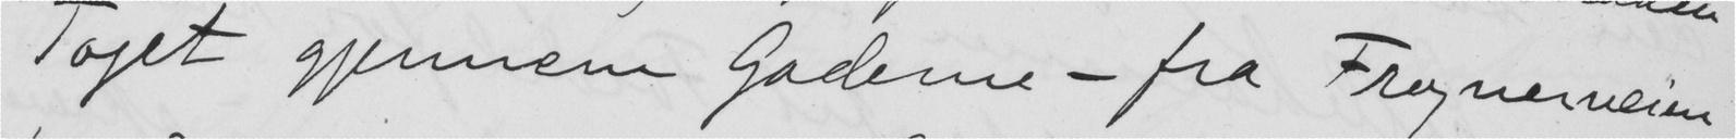Toget gjennem Gaderne - fra Frognerveien
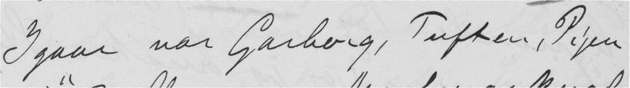Igaar var Garborg, Tuften, Pigen
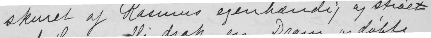skuret af Rasmus egenhændig og strøet
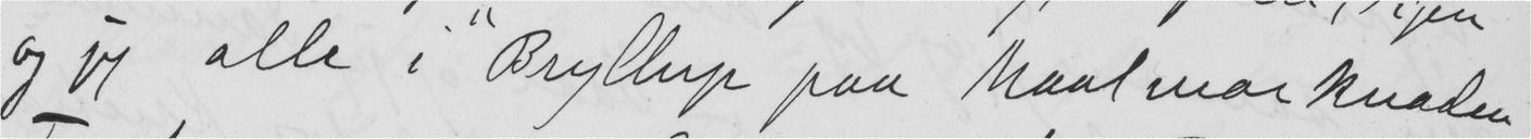og jeg alle i Bryllug paa Maalmarknaden.
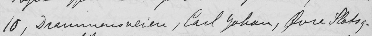10, Drammensveien, Carl Johan, Øvre Slotsg.
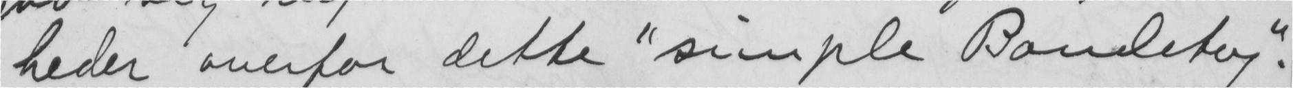heder overfor dette "simple Bondetog".
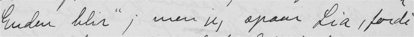Enden blir"; men jeg spaar Lia, fordi
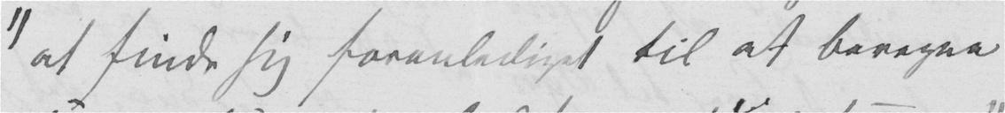"at finde sig foranlediget til at beregne
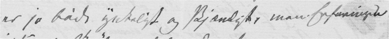er jo både ynkeligt og skjændigt, men Erfaringerne
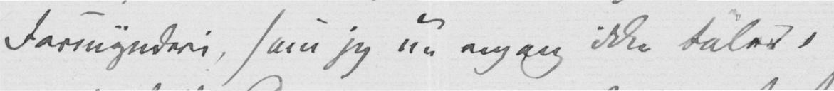Formynderi, som jeg nu engang ikke tåler,
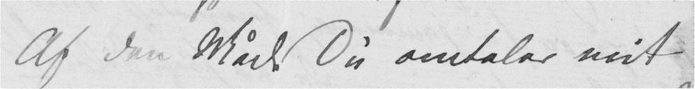Af den Måde Du omtaler mit
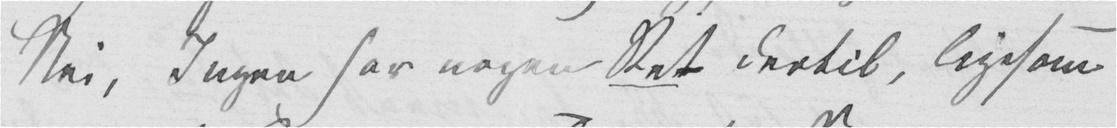Nei, Ingen har nogen Ret dertil, ligesom
